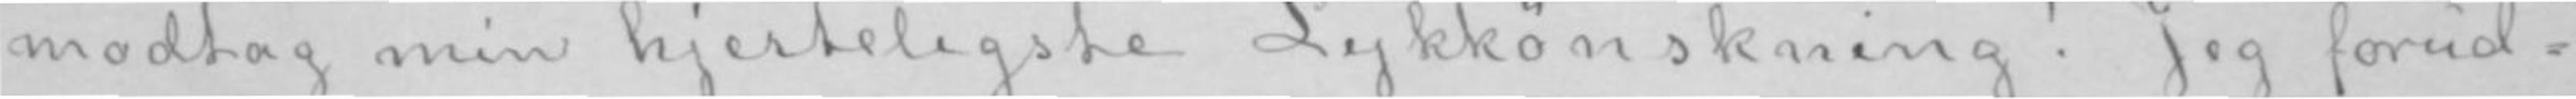modtag min hjerteligste Lykkønskning! Jeg forud-
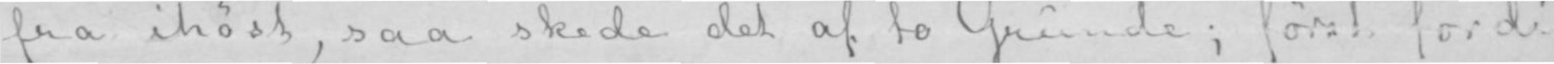fra ihøst, saa skede det af to Grunde; først fordi
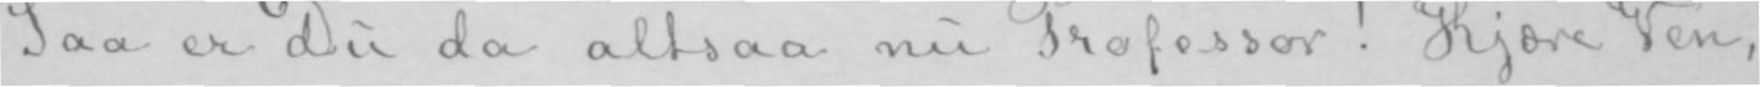Saa er Du da altsaa nu Professor! Kjære Ven,
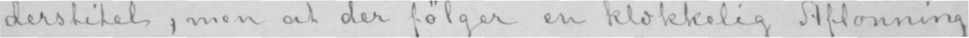derstitel, men at der følger en klækkelig Aflønning
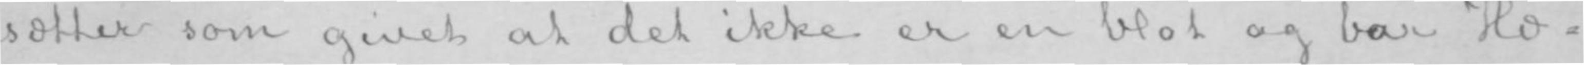sætter som givet at det ikke er en blot og bar Hæ-
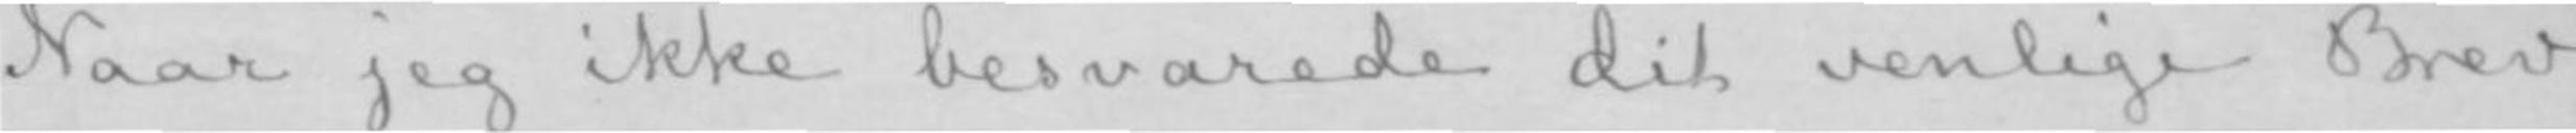Naar jeg ikke besvarede dit venlige Brev
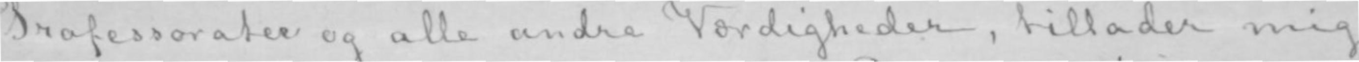Professorater og alle andre Værdigheder, tillader mig
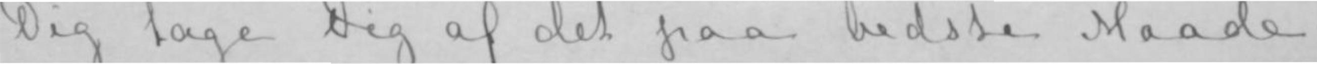Dig tage Dig af det paa bedste Maade.
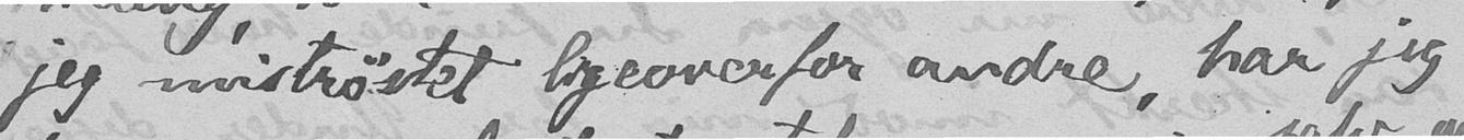jeg mistrøstet ligeoverfor andre, har jeg
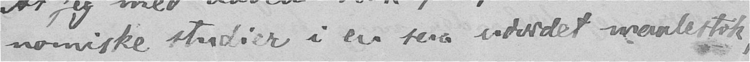nomiske studier i en saa udvidet maalestok,
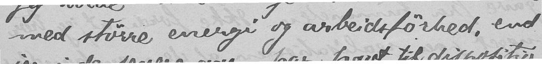med større energi og arbeidsførhed, end
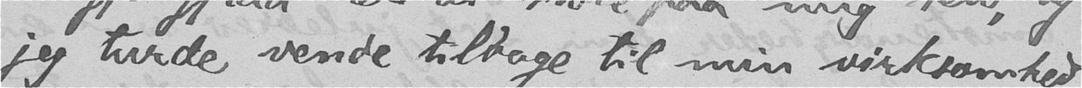jeg turde vende tilbage til min virksomhed
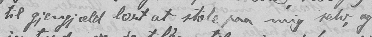til gjengjæld lært at stole paa mig selv, og
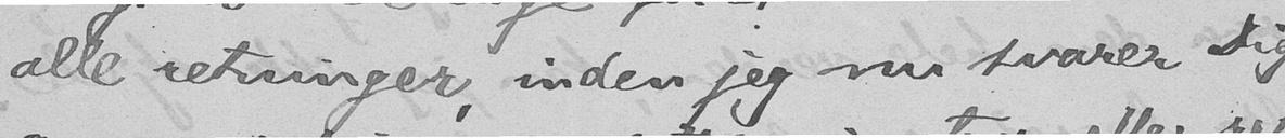alle retninger, inden jeg nu svarer Dig.
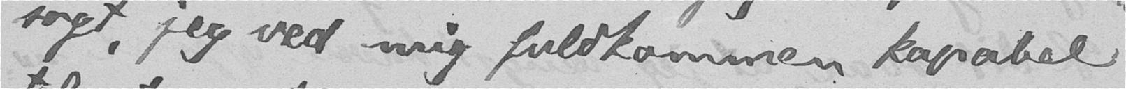sagt, jeg ved mig fuldkommen kapabel
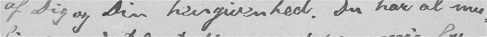af Dig og Din hengivenhed. Du har al mu-
